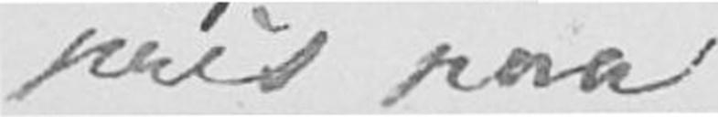pris paa.
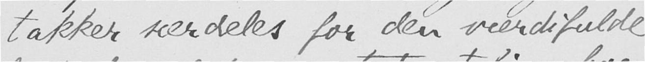takker særdeles for den værdifulde
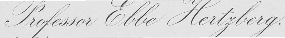Professor Ebbe Hertzberg
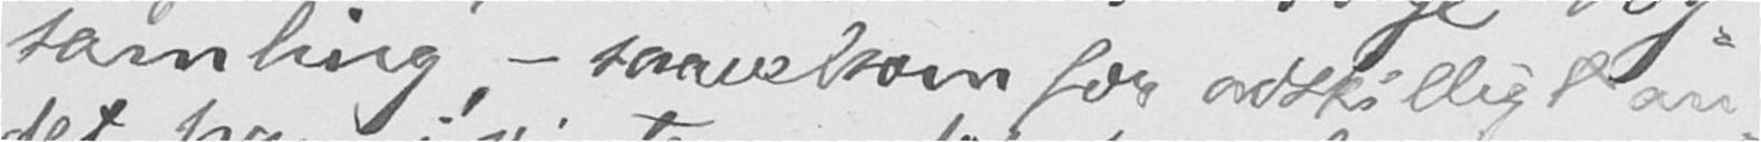samling - saavelsom for adskilligt an-
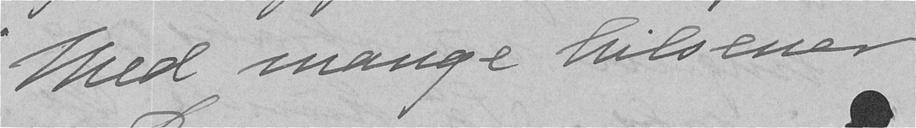Med mange hilsener
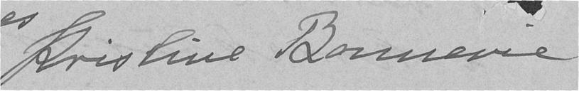Kristine Bonnevie
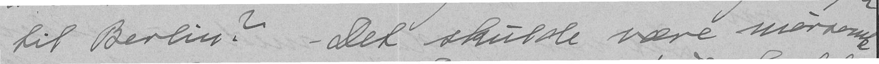til Berlin? - Det skulde være morsomt
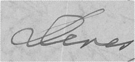Deres
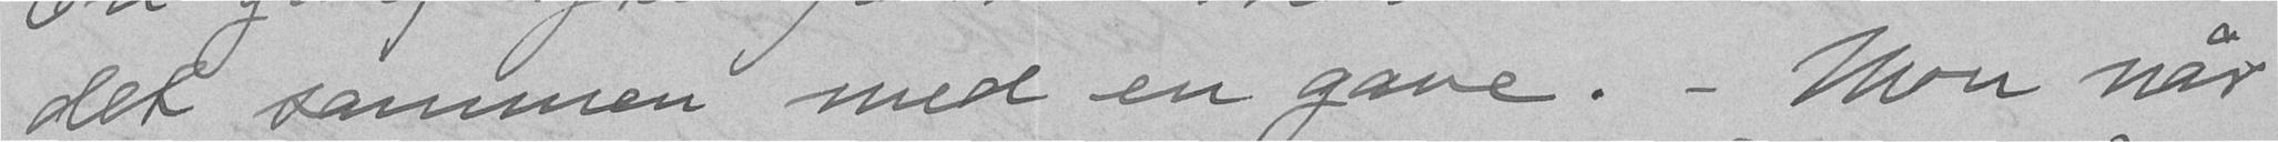det sammen med en gave. - Mon når
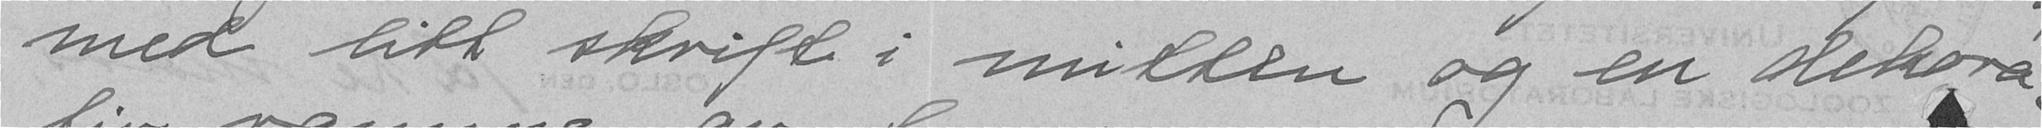med litt skrift i mitten og en dekora-
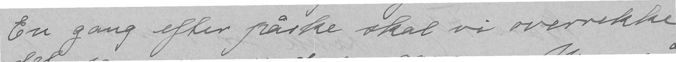En gang efter påske skal vi overrekke
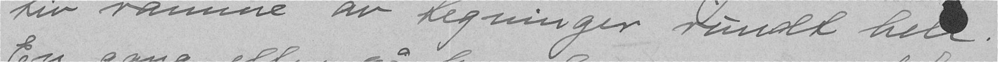tiv ramme av tegninger rundt hele.
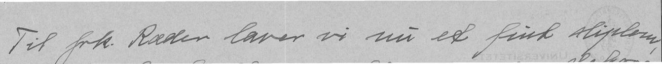Til frk. Ræder laver vi nu et fint diplom
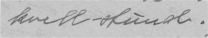kvell-stund.
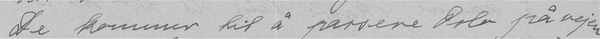De kommer til å passere Oslo på vejen
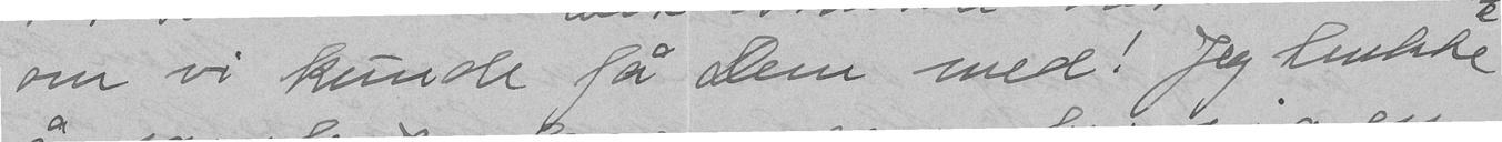om vi kunde få Dem med! Jeg tenkte
